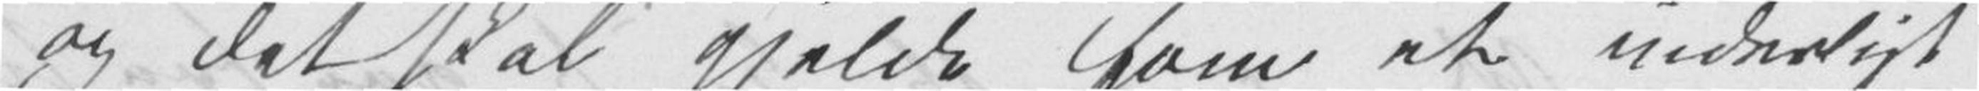og det skal gjelde som et inderligt
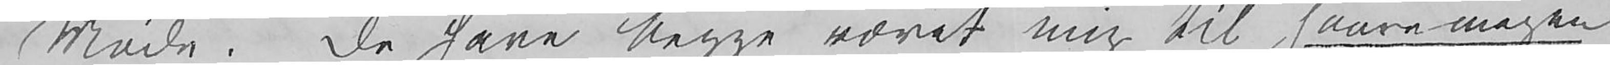Møde. De have begge været mig til saare megen
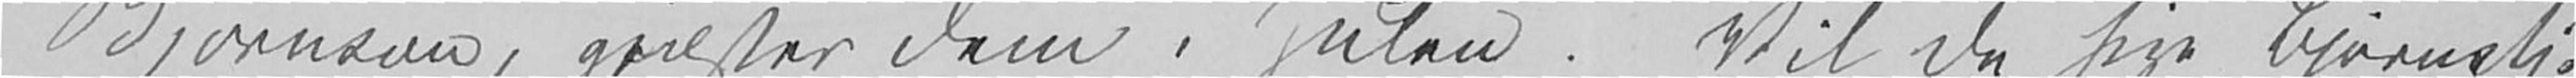Bjørnson, gjæster Dem i Julen. Vil De sige Bjørnstj.
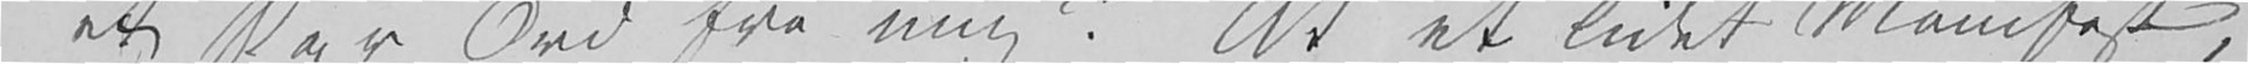et Par Ord fra mig? At et lidet Manifest,
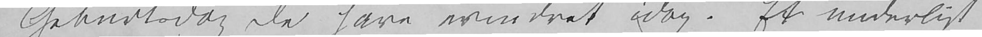Geburtsdag de have erindret idag. Et underligt
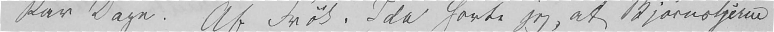Par Dage. Af Frøk. Ida hørte jeg, at Bjørnstjerne
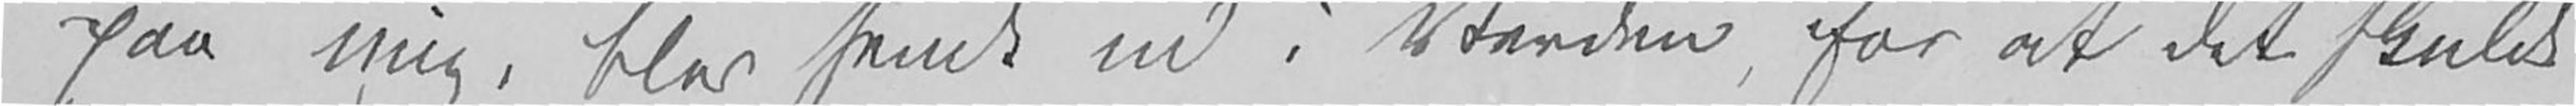paa mig, blev sendt ud i Verden, for at det skulde
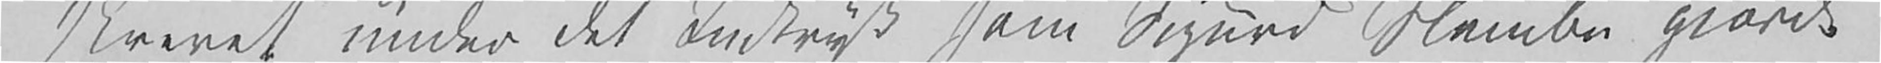skrevet under det Indtryk som «Sigurd Slembe» gjorde
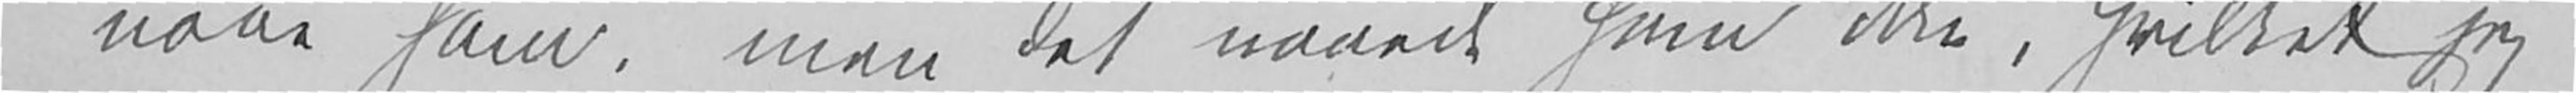naae ham, men det naaede ham ikke, hvilket jeg
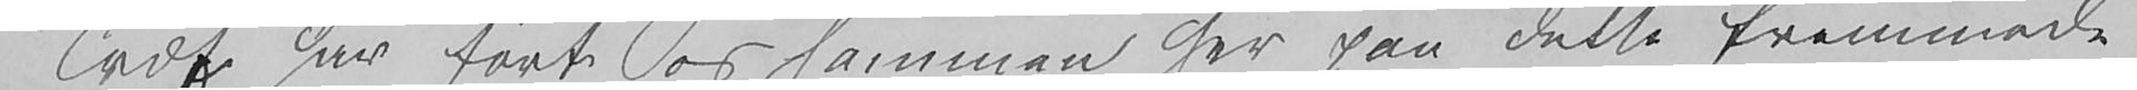Træf har ført os sammen her paa dette fremmede
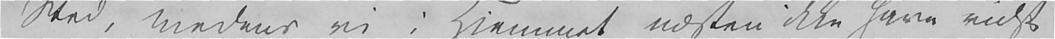Sted, medens vi i Hjemmet næsten ikke have vidst
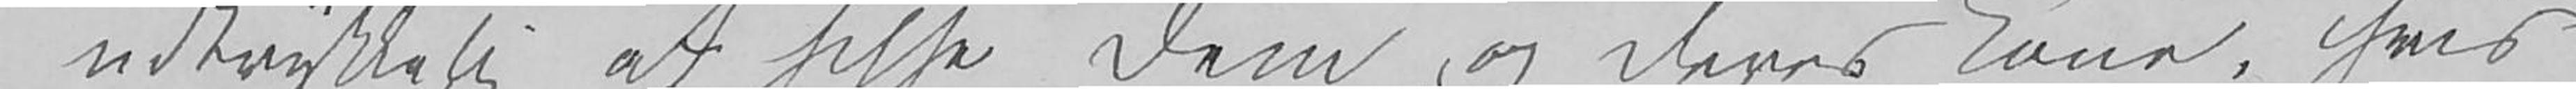udtrykkelig at hilse Dem og Deres Kone, hvis
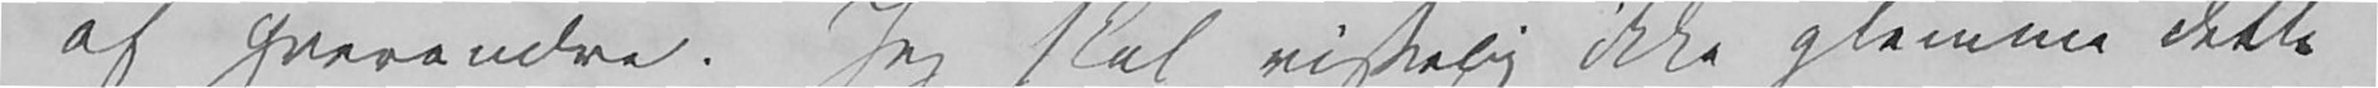af hverandre. Jeg skal visselig ikke glemme dette
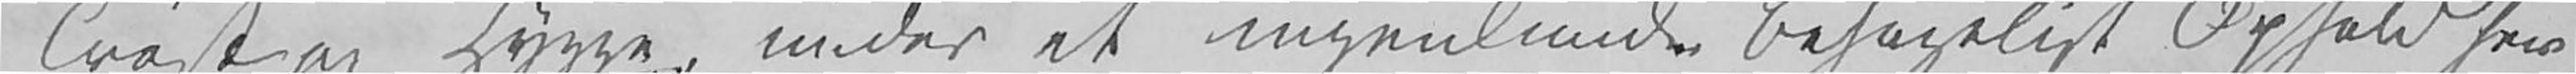Trøst og Hygge, under et ingenlunde behageligt Ophold her
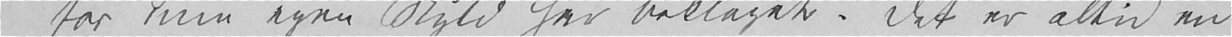for min egen Skyld har beklaget. Det er altid en
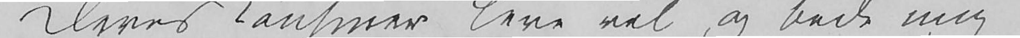Deres Cousiner leve vel og bede mig
